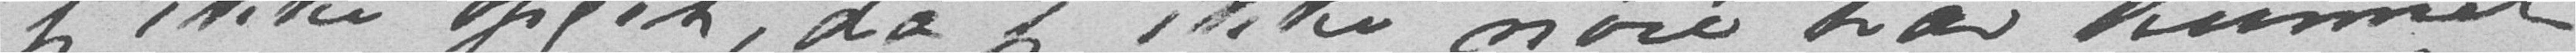jeg ikke opgit, da jeg ikke nøie har kunnet
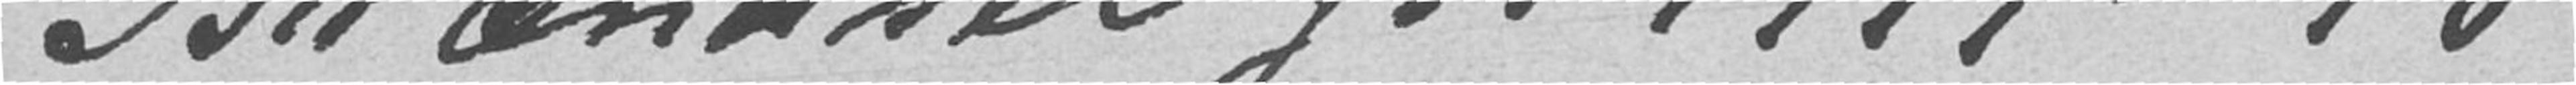Brændsel for 1917-18
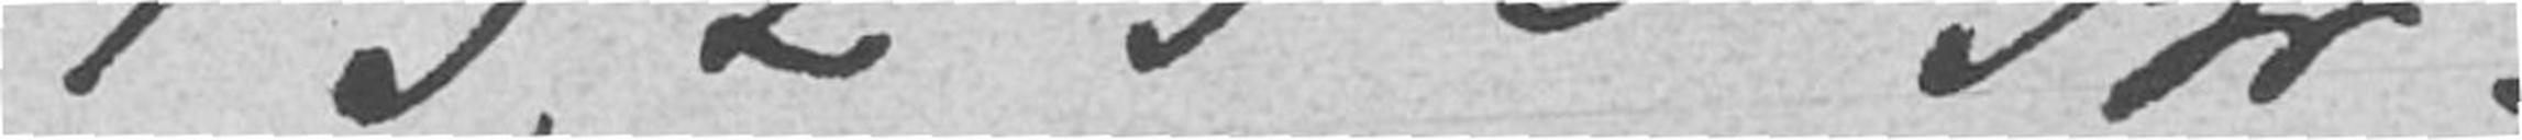13,250 Kr
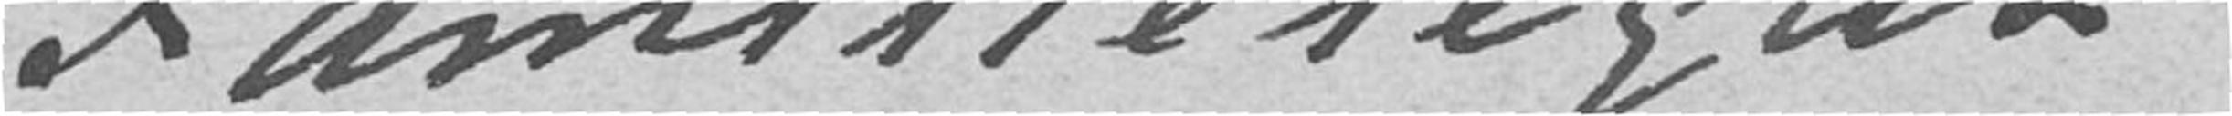Familielegat
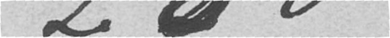200
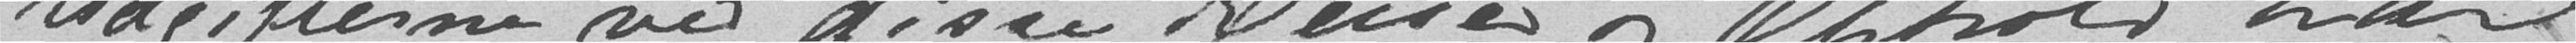Udgifterne ved disse Reiser og Ophold har
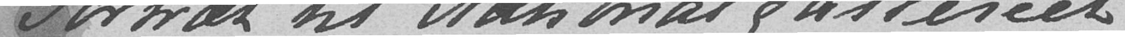Portrært til Nationalgalleriet
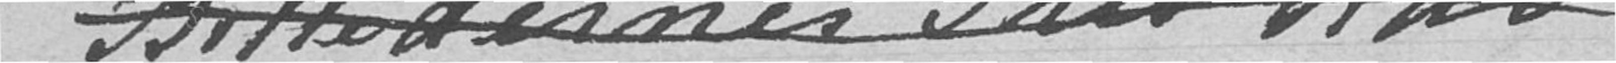Billedernes Pris har
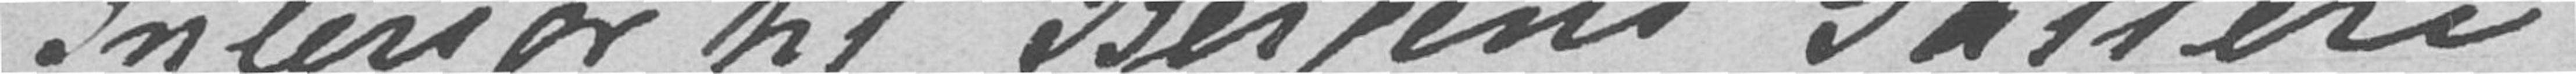Interiør til Bergens Galleri
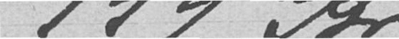199 Kr
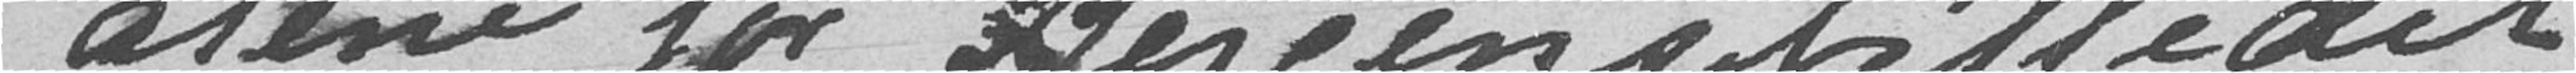alene for Bergensbilledet
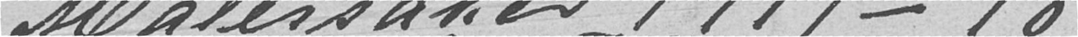Malersaker 1917-18
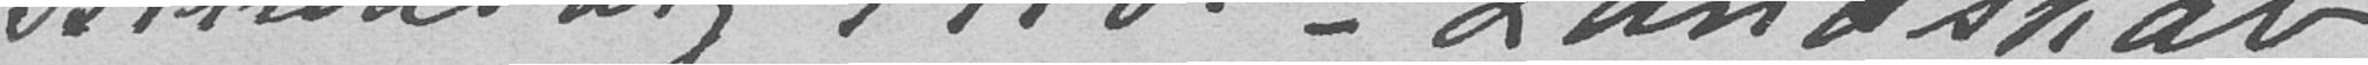Billedsalg 1918. - Landskab
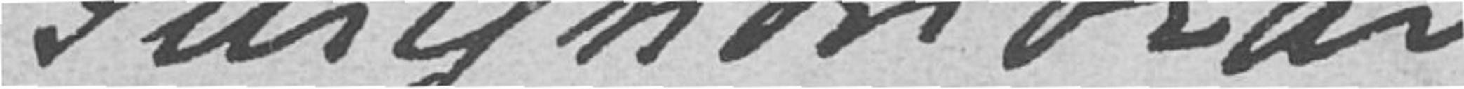Juryhonorar
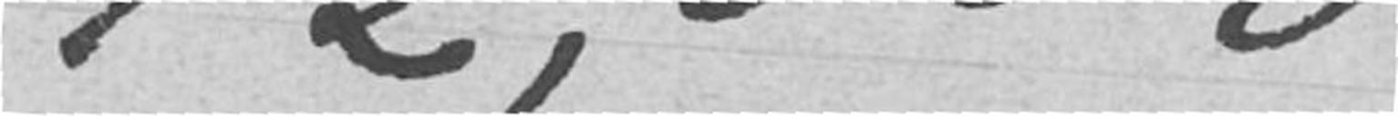12000
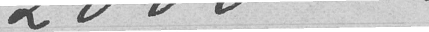2000
